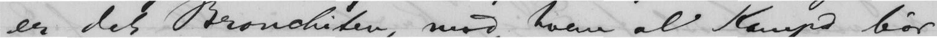er det Bronchiten, mod hvem al Kamp bør
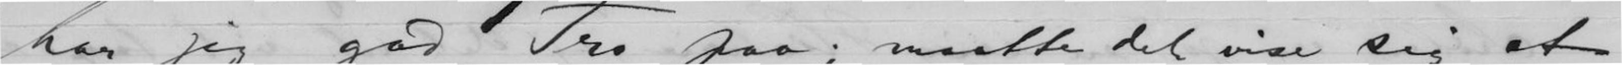har jeg god Tro paa; maatte det vise sig at
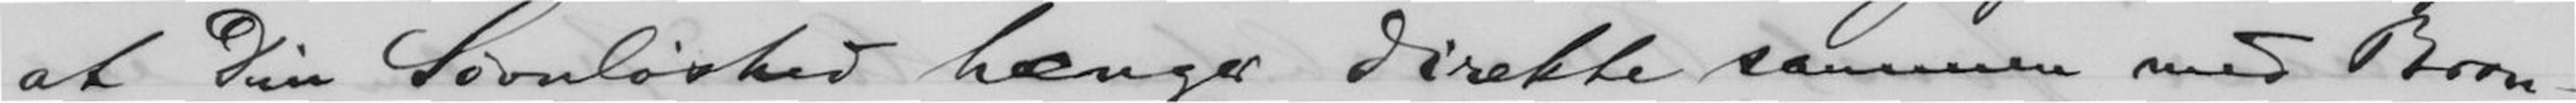at Din Søvnløshed hænger direkte sammen med Bron-
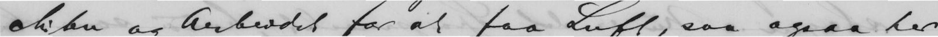chiten og Arbeidet for at faa Luft, saa ogsaa her
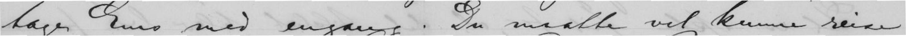tage Ems med engang. Du maatte vel kunne reise
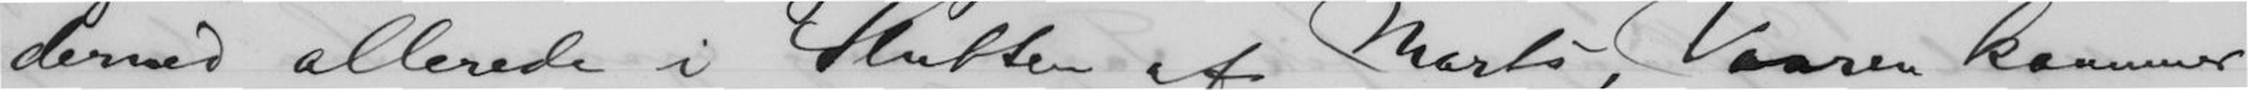derned allerede i Slutten af Marts, Vaaren kommer
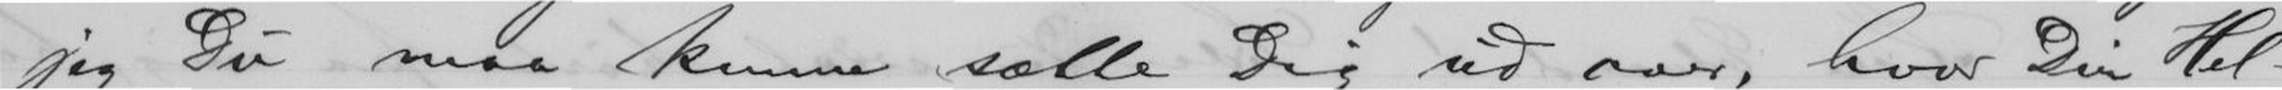jeg Du maa kunne sætte Dig ud over, hvor Din Hel-
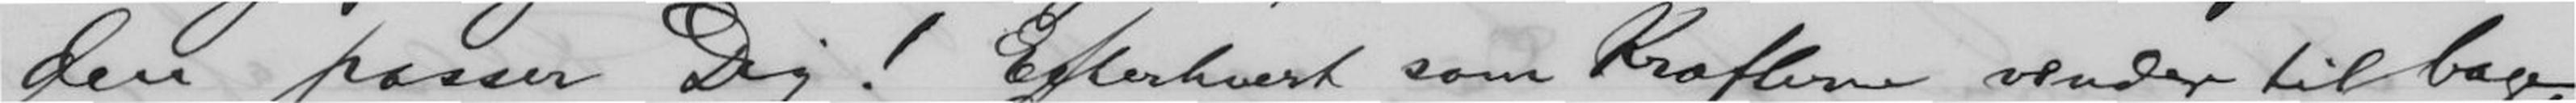den passer Dig! Efterhvert som Kraften vender tilbage,
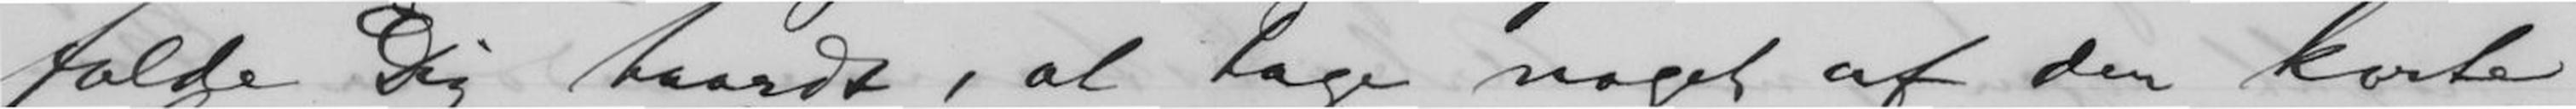falde Dig haardt, at tage noget af den korte
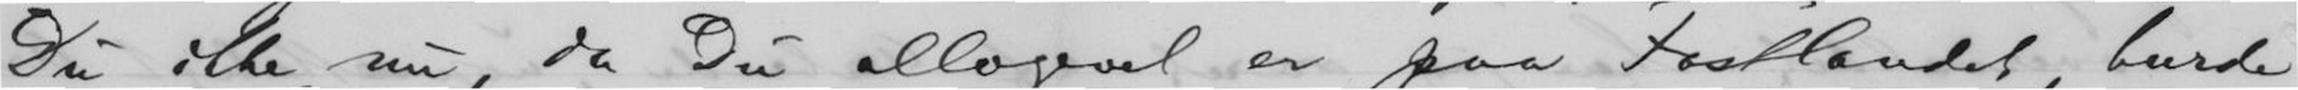Du ikke nu, da Du alligevel er paa Fastlandet, burde
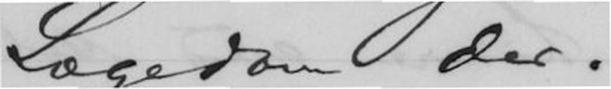Lægedom der.
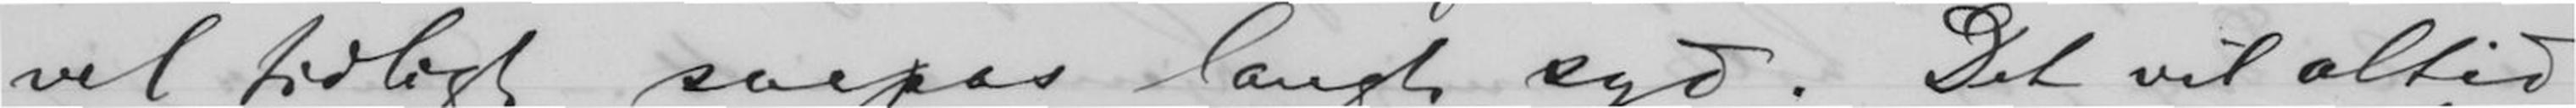vel tidligt saapas Langt syd. Det vil altid
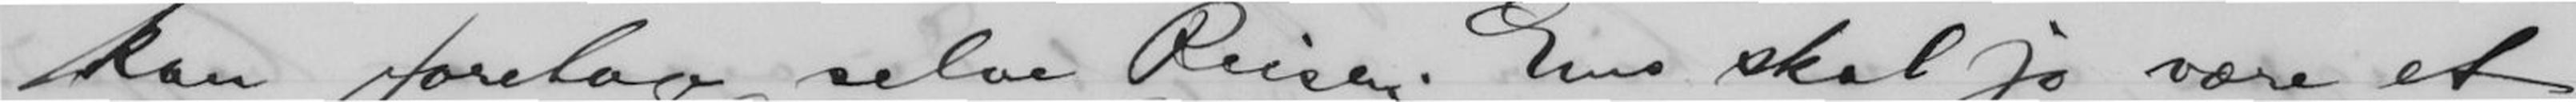kan foretage selve Reisen. Ems skal jo være et
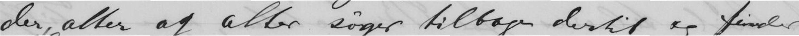der, atter og atter søger tilbage dertil og finder
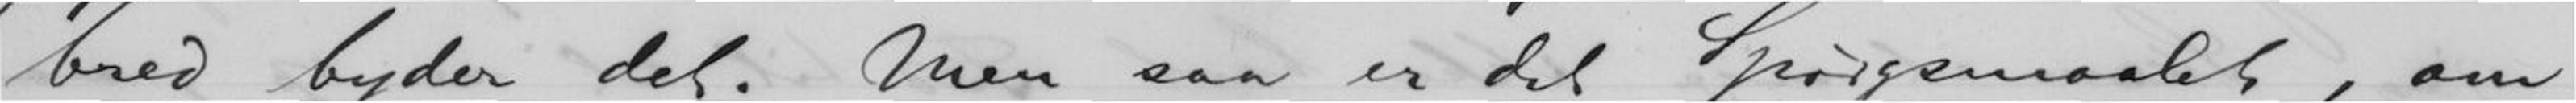bred byder det. Men saa er det Spørgsmaaalet, om
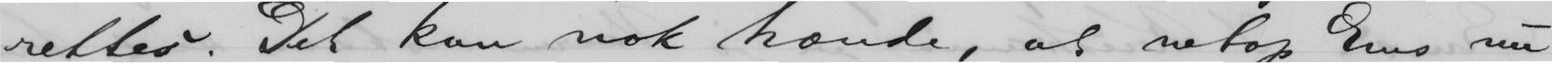rettes. Det kan nok hænde, at netop Ems nu
